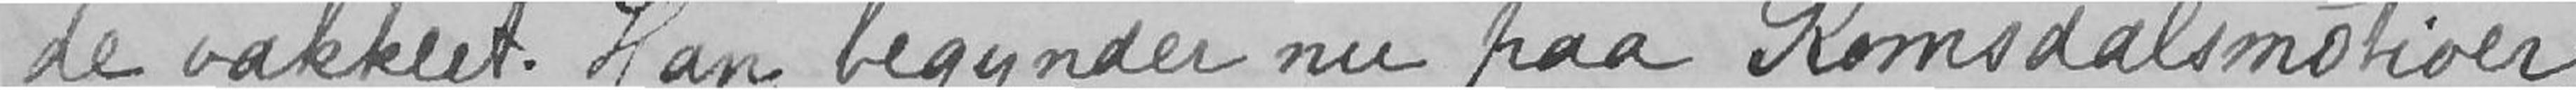de vakkert. Han begynder nu paa Romsdalsmotiver,
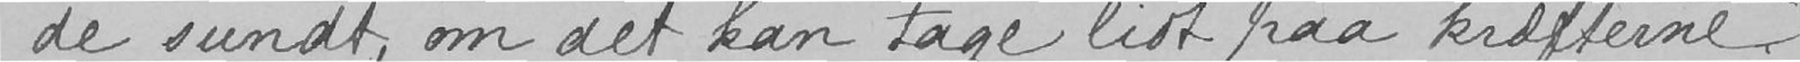de sundt, om det kan tage lidt paa kræfterne.
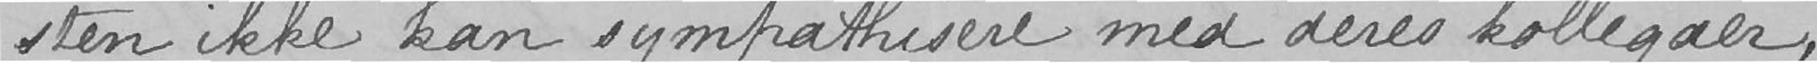sten ikke kan sympathisere med deres kollegaer,
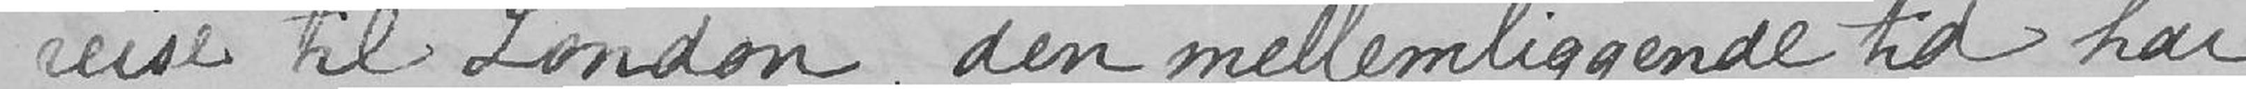reise til London, den mellemliggende tid har
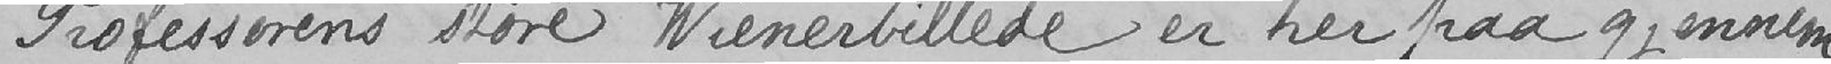Professorens store Wienerbillede er her paa gjennem-
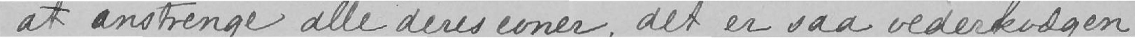at anstrenge alle deres evner, det er saa vederkvægen
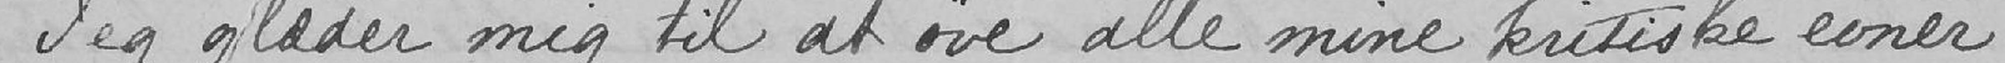Jeg glæder mig til at øve alle mine kritiske evner
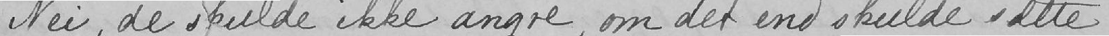Nei, de skulde ikke angre, om det end skulde sætte
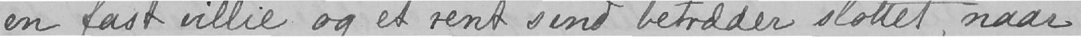en fast villie og et rent sind betræder slottet, naar
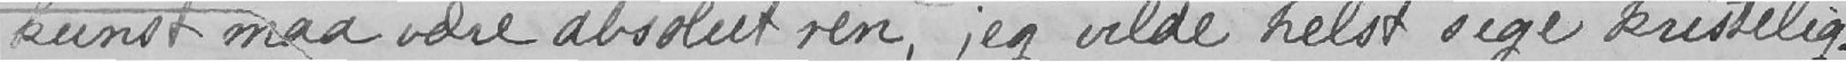kunst maa være absolut ren, jeg vilde helst sige kristelig.
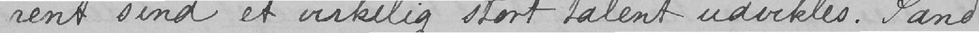rent sind et virkelig stort talent udvikles. Sand
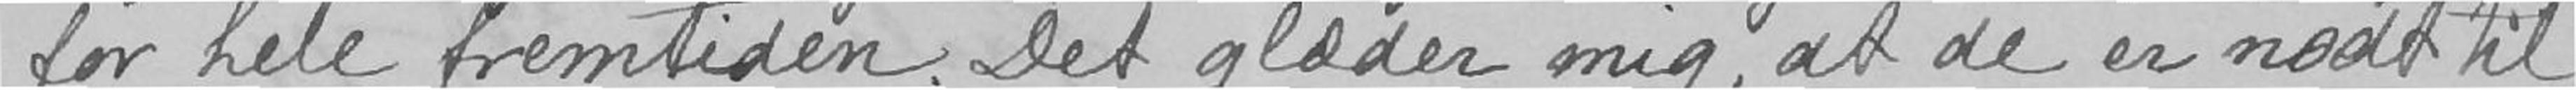for hele fremtiden. Det glæder mig, at de er nødt til
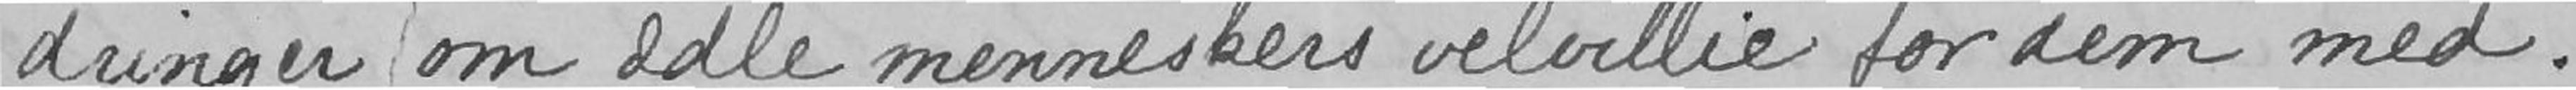dringer om ædle menneskers velvillie for dem med.
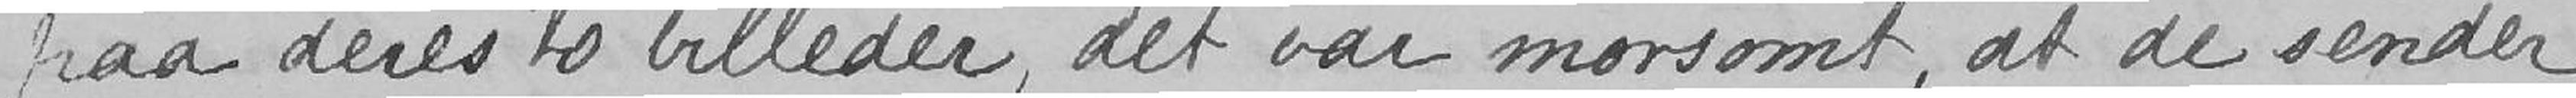paa deres to billeder, det var morsomt, at de sender
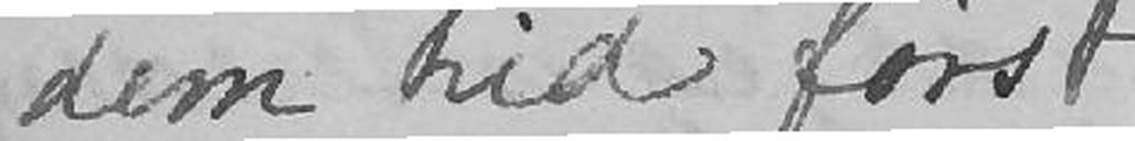dem hid først.
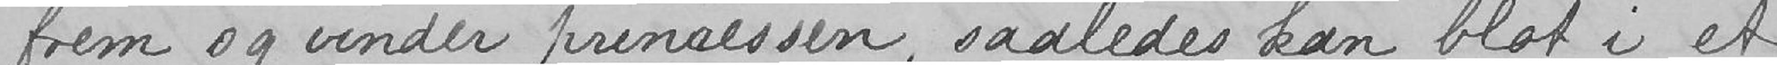frem og vinder prinsessen, saaledes kan blot i et
